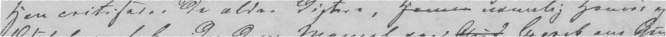Han critiserer de ældre Digtere, Homer nemlig Homer og
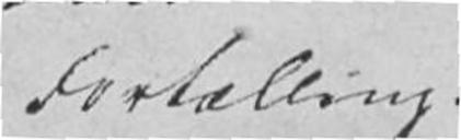Fortælling.
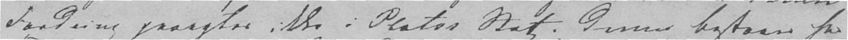Fordring paaagtes ikke i Platos Stat. Denne bestaae for
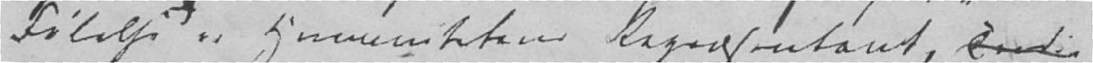Følelse er Humanitetens Repræsentant,
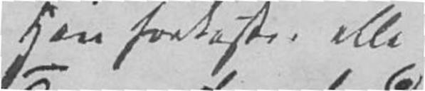Han forkaster alle
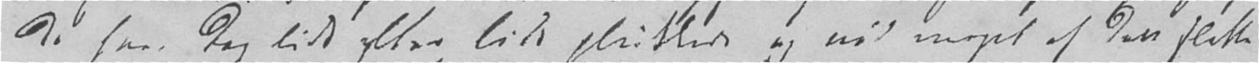de have dog lidt efter lidt plukkede og nød noget af den slette
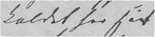Kaldet for sit
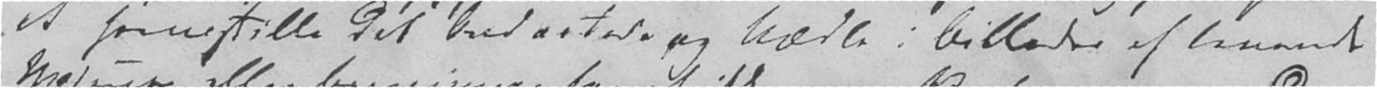at fremstille det Ondartede og Uædle i Billeder af levende
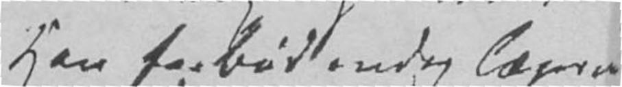Han forbød endog Læger
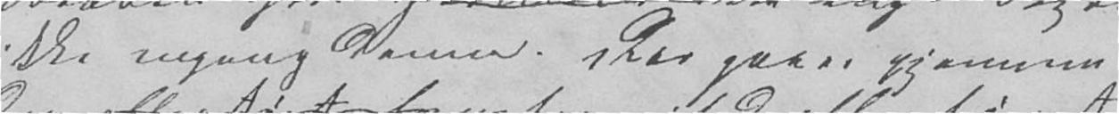ikke engang denne. Der gaaer gjennem
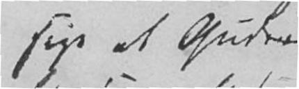sige at Guder
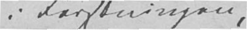i Forskningen,
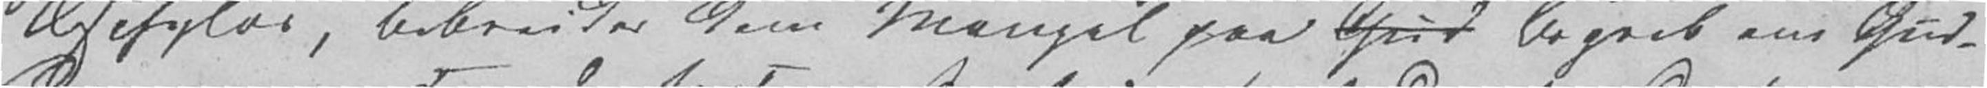Æschylos, bebreider dem Mangel paa Gud Begreb om Gud-
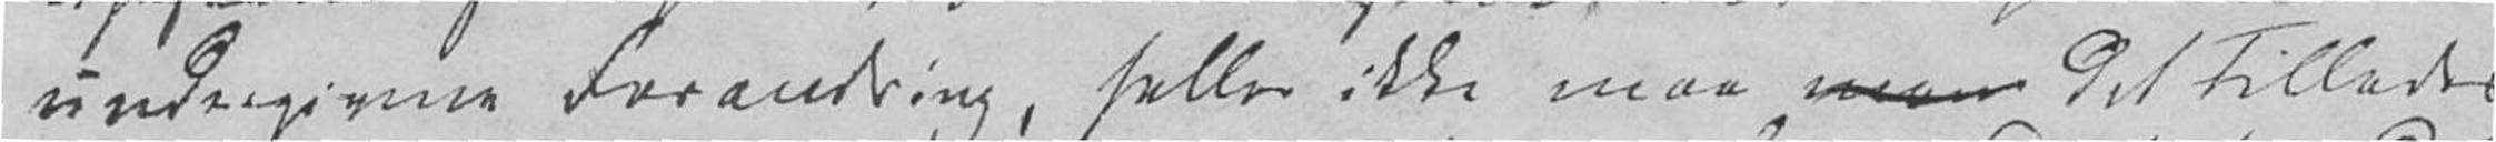undergivne Forandring, heller ikke maa man det tillades
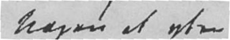Nogen at ytre
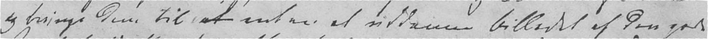og bringe dem til at enten at uddanne Billedet af den gode
